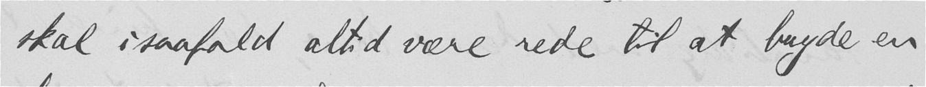skal isaafald altid være rede til at bryde en
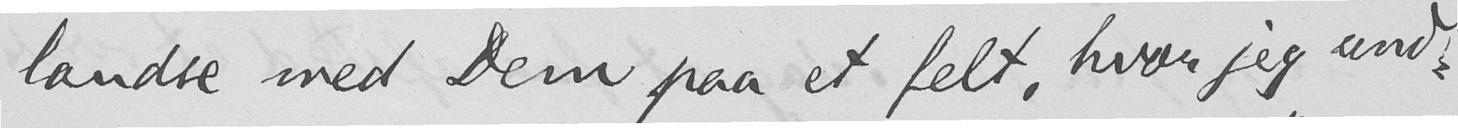landse med Dem paa et felt, hvor jeg und-
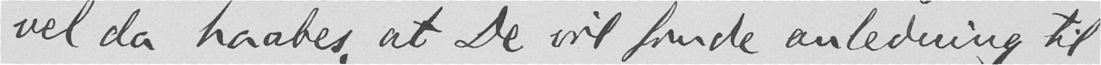vel da haabes, at De vil finde anledning til
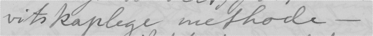vitskaplege methode –
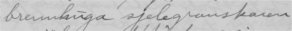brennhuga sjelegranskaren
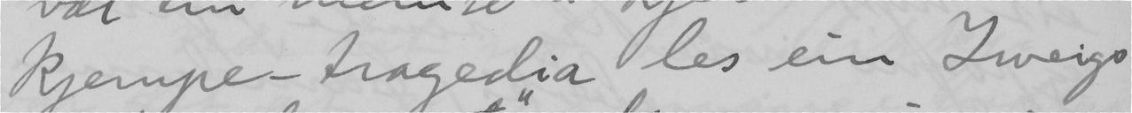kjempe-tragedia les ein Zweigs
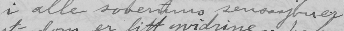i alle soverums sensasjoner
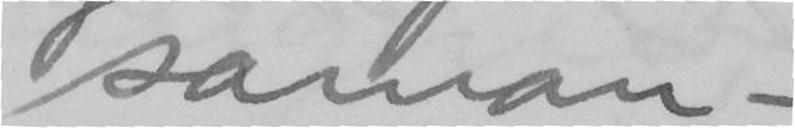saman –
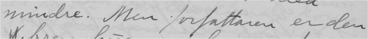mindre. Men forfattaren er den
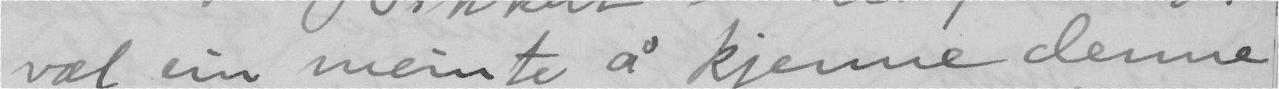væl ein meinte å kjenne denne
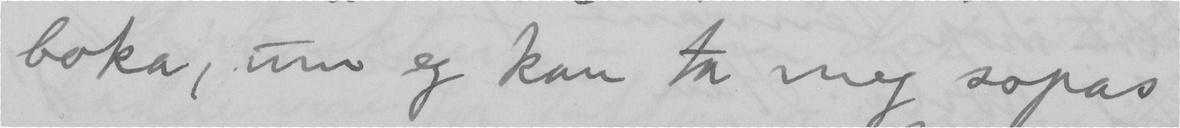boka, um eg kan ta meg sopas
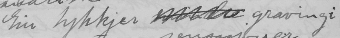Ein tykkjer  gravingi
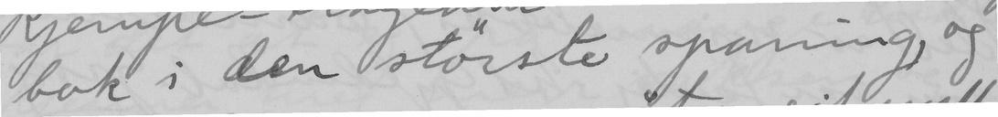bok i den störste spaning, og
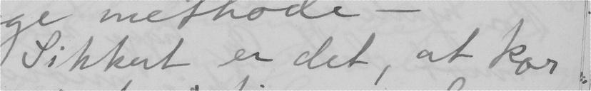Sikkert er det, at kor
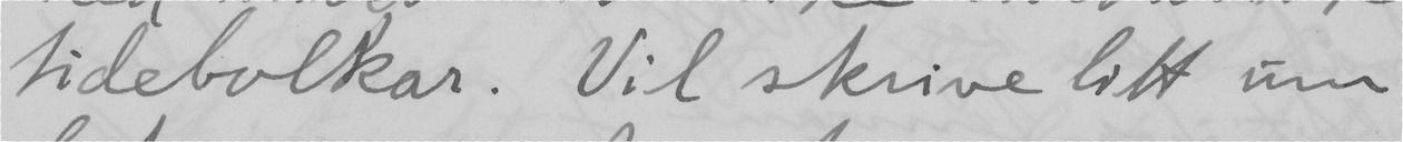tidebolkar. Vil skrive litt um
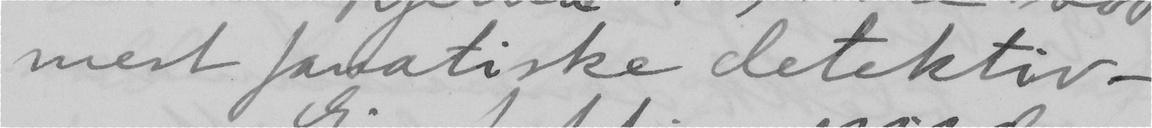mest fanatiske detektiv –
